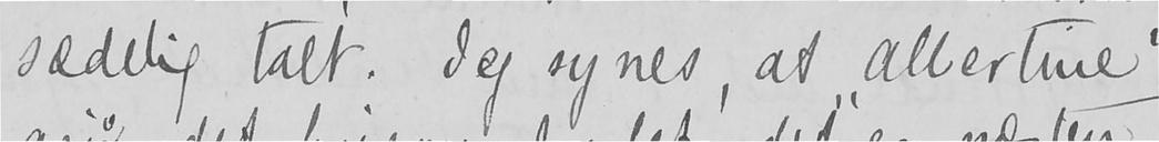sædelig talt. Jeg synes, at "Albertine"
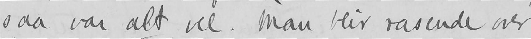saa var alt vel. Man blir rasende over
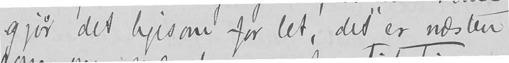gjør det ligesom for let, det er næsten
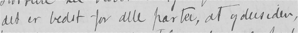det er bedst for alle parter, at ydersiden,
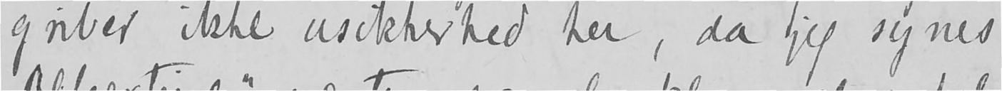griber ikke usikkerhed her, da jeg synes
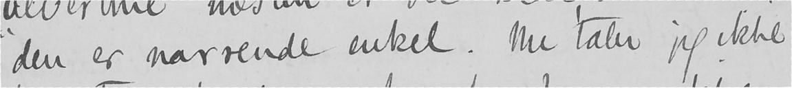den er narrende enkel. Nu taler jeg ikke
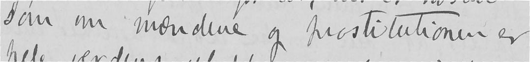som om mændene og prostitutionen er
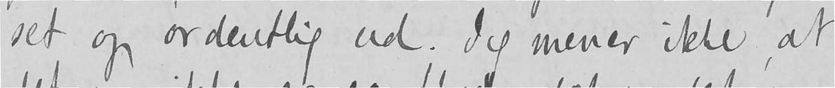set og ordentlig ud. Jeg mener ikke, at
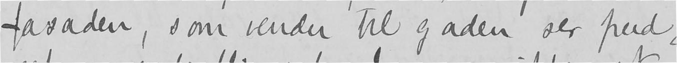fasaden, som vender til gaden ser pud-
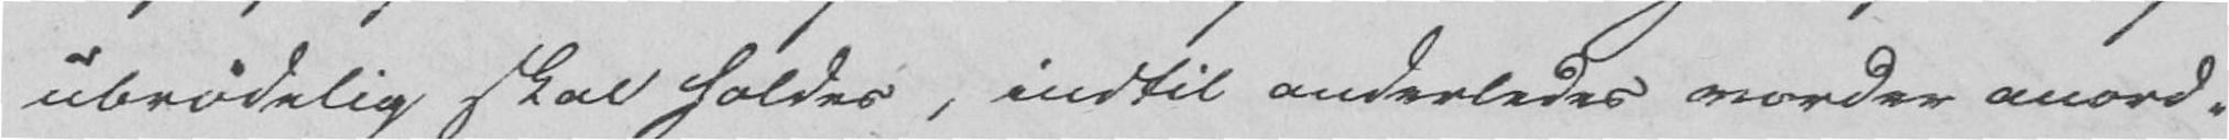ubrødelig skal holdes, indtil anderledes vorder anord-
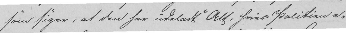som siger, at den har udeladt "Alt, hvis Politien e-
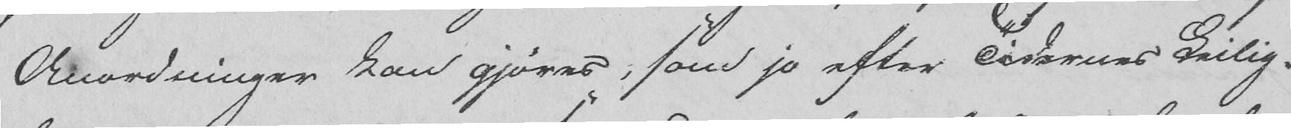Anordninger kan gjøres, som jo efter Tidernes Leilig-
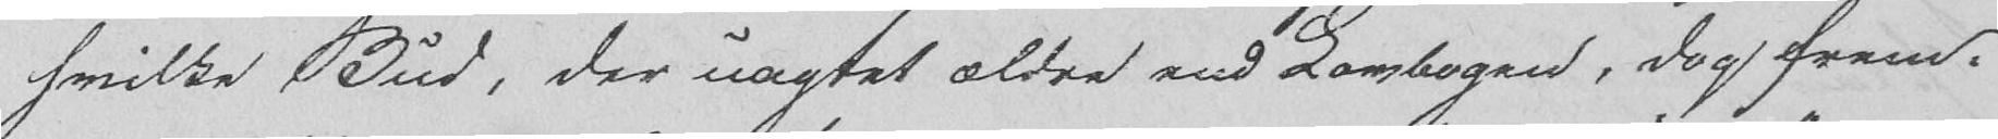hvilke Bud, der uagtet ældre end Lovbogen, dog frem-
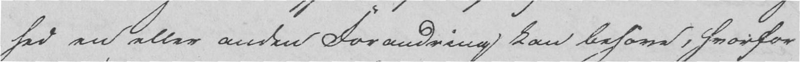hed en eller anden Forandring kan behøve, hvorfor
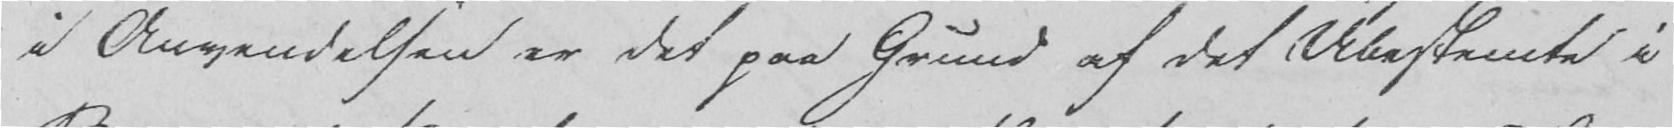i Anvendelsen er det paa Grund af det Ubestemte i
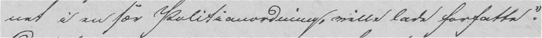net i en sær Politianordning, ville lade forfatte".
